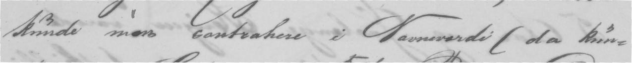kunde man contrahere i Navneværdi (da kun-
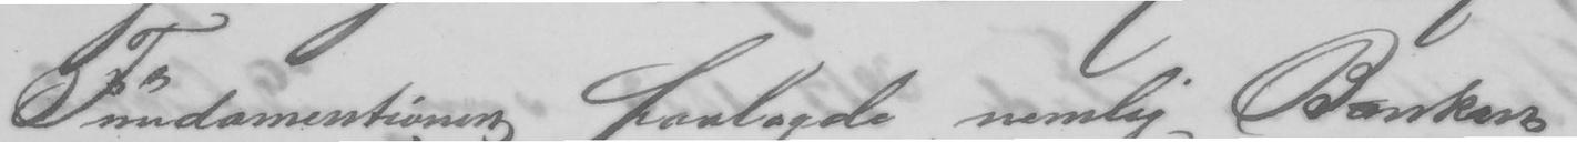Fundamentionen paalagde nemlig Banken
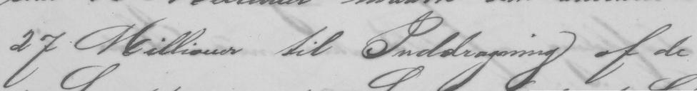27 Millioner til Inddragning af de
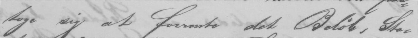tage sig at forrente det Beløb, Sta-
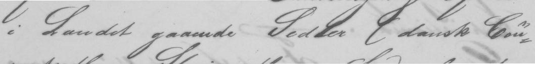i Landet gaaende Sedler (dansk Cou-
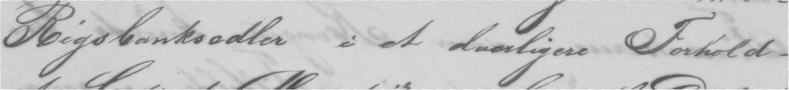Rigsbanksedler i et daarligee Forhold -
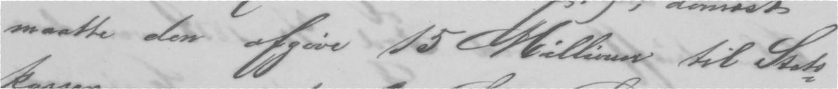maatte den afgive 15 Millioner til Stats-
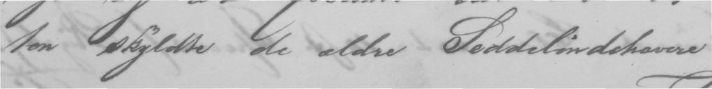ten skyldte de ældre Seddelindehavere
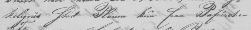keligvis stod Planen kun paa Papiret. -
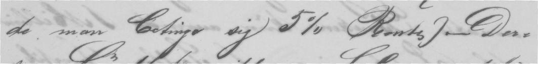de man betinge sig 5% Rente). Der-
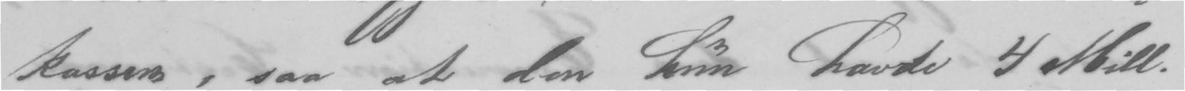kassen, saa at den kun havde 4 Mill.
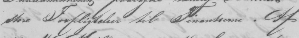store Forpligtelser til Finantserne. Af
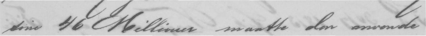sine 46 Millioner maatte den anvende
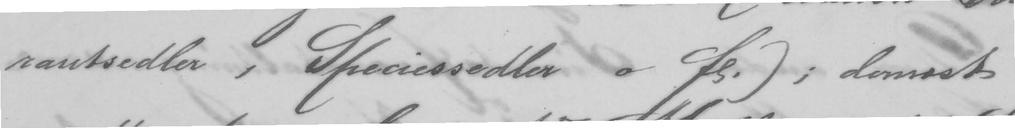rantsedler, Speciessedler a fl.); dernest
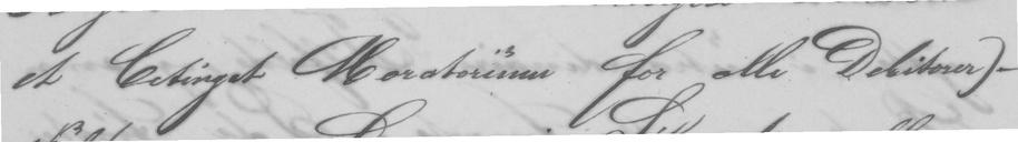et betinget Moratorium for alle Debitorer) -
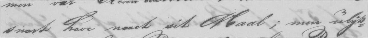snart have naaet sit Maal; men ulyk-
